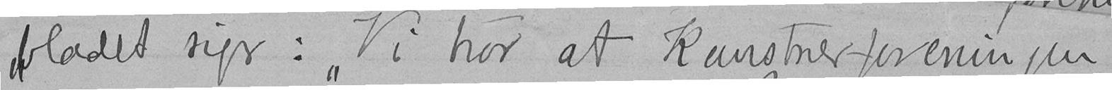bladet siger: "Vi tror at Kunstnerforeningen
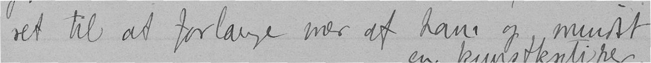ret til at forlange mer af ham og mindst
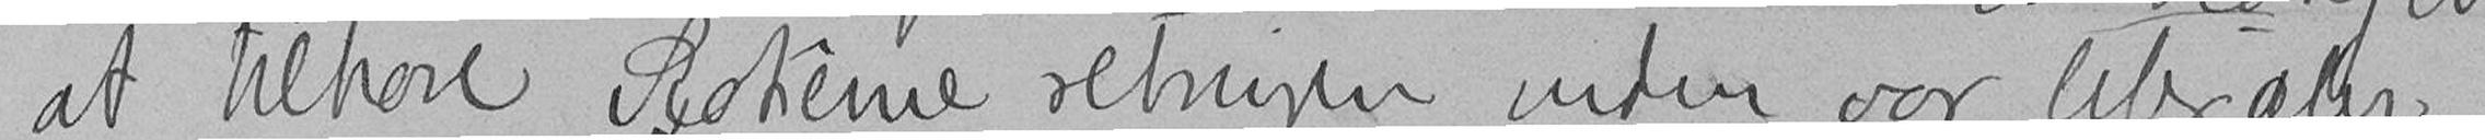at tilhøre Bohême retningen inden vor literatur
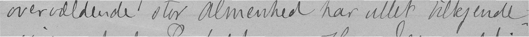overvældende stor Almenhed har villet tilkjende-
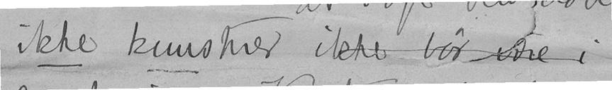ikke kunstner ikke bør være i
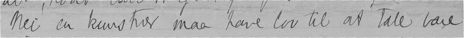Nei en kunstner maa have lov til at tale bare
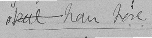skal han høre
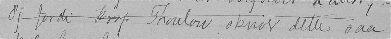Og fordi prof. Thaulow, skriver dette saa
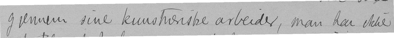gjennem sine kunstneriske arbeider, man har ikke
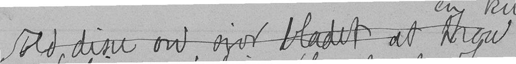ved disse ord siger bladet at Thow
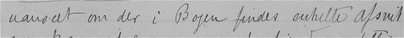uanseet om der i Bogen findes enkelte Afsnit
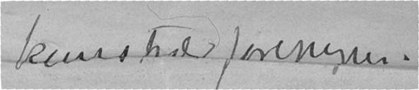kunstnerforeningen.
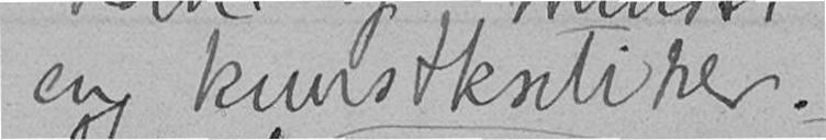en kunstkritiker.
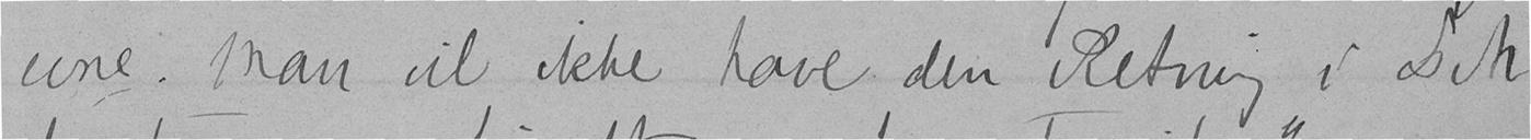evne. Man vil ikke have den Retning i Lit-
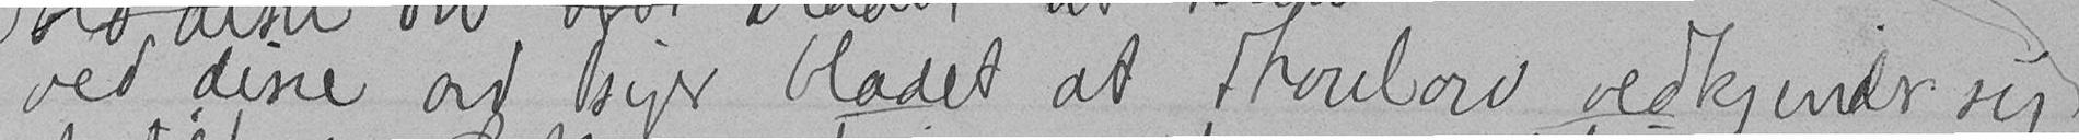ved disse ord siger, bladet at Thaulow vedkjender sig
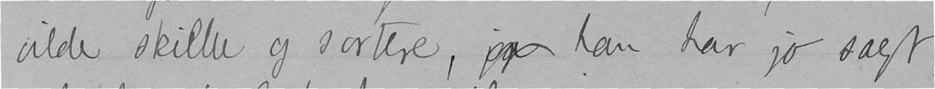vilde skille og sortere, og han har jo sagt
